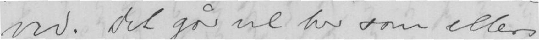ved. Det går vel her som ellers
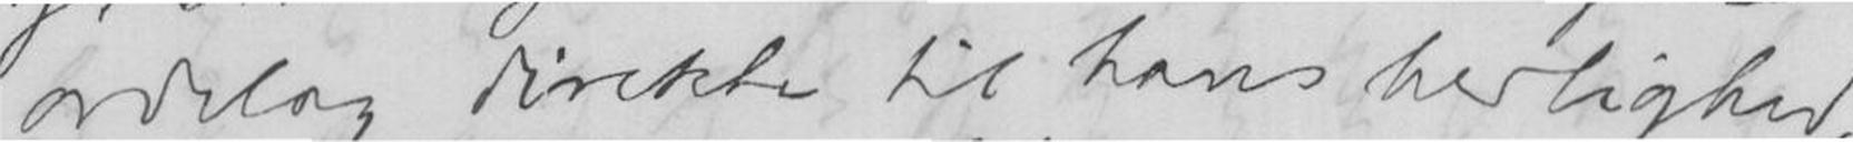ordlag direkte til hans herlighed,
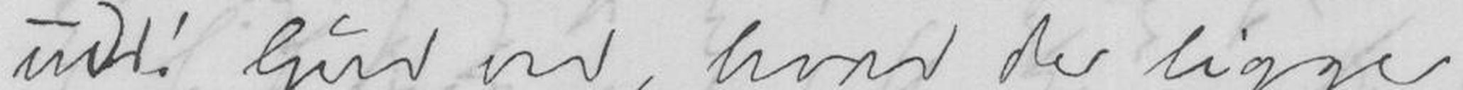ud! Gud ved, hvad der ligger
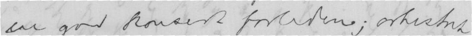en god konsert forleden; orkestret
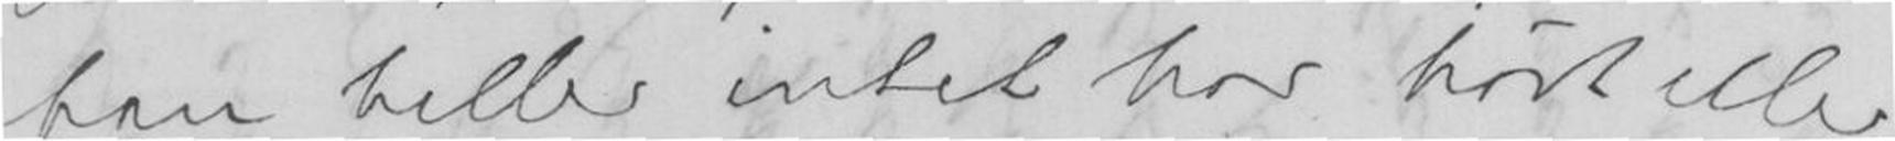han heller intet har hørt eller
